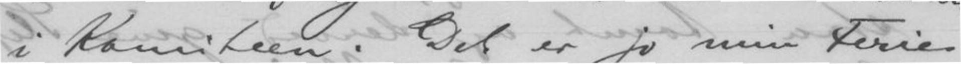i Komiteen. Det er jo min Ferie-
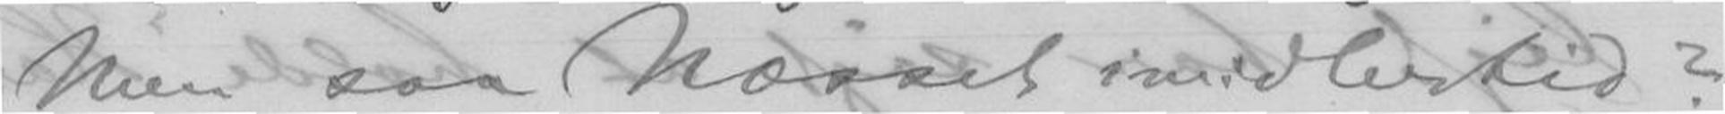Men saa Næsset imidlertid?
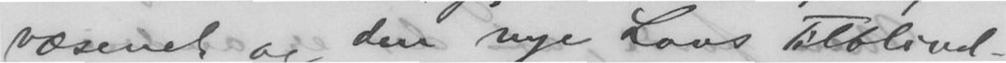væsenet og den nye Lovs Tilblivel-
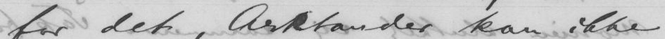for det, Arctander kan ikke
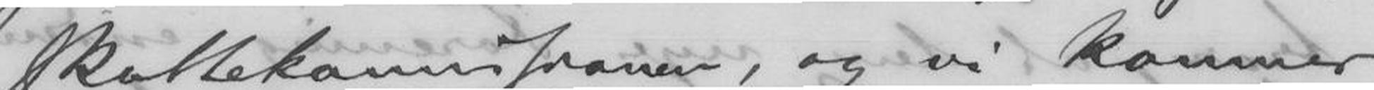skattekommisionen, og vi kommer
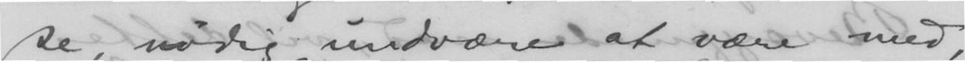se, nødig undvære at være med,
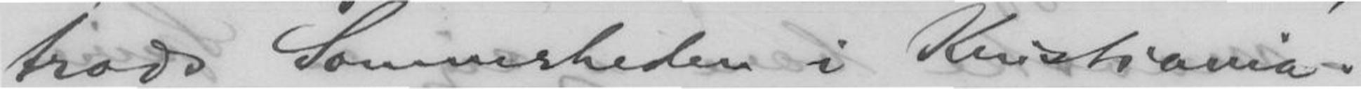trods Sommerheden i Kristiania.
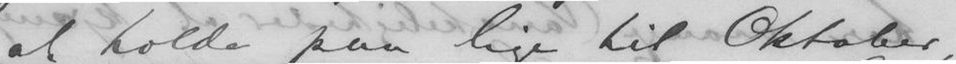at holde paa lige til Oktober,
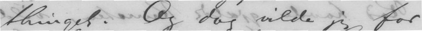thinget. Og dog vilde jeg for
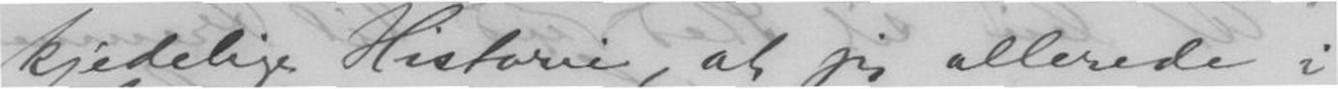kjedelige Historie, at jeg allerede i
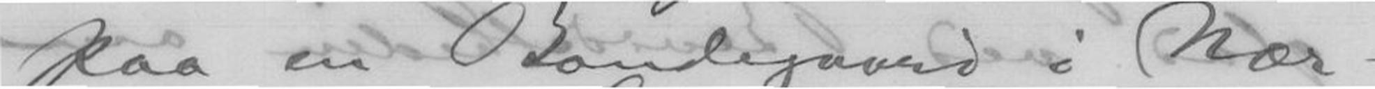paa en Bondegaard i Nær-
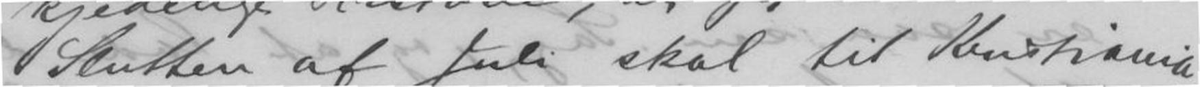Slutten af Juli skal til Kristiania
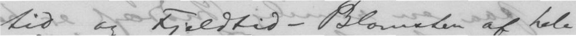tid og Fjeldtid - Blomsten af hele
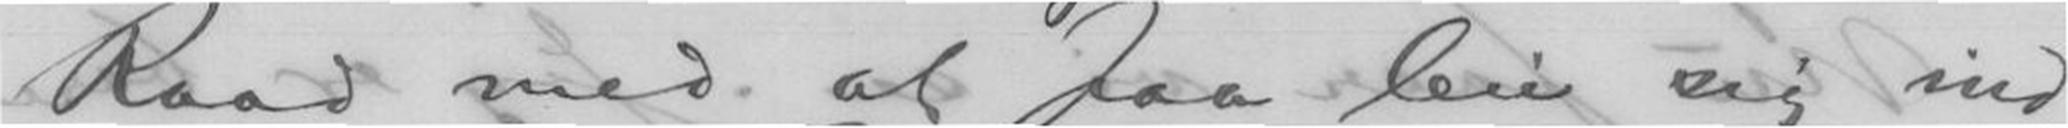Raad med at faa leie sig ind
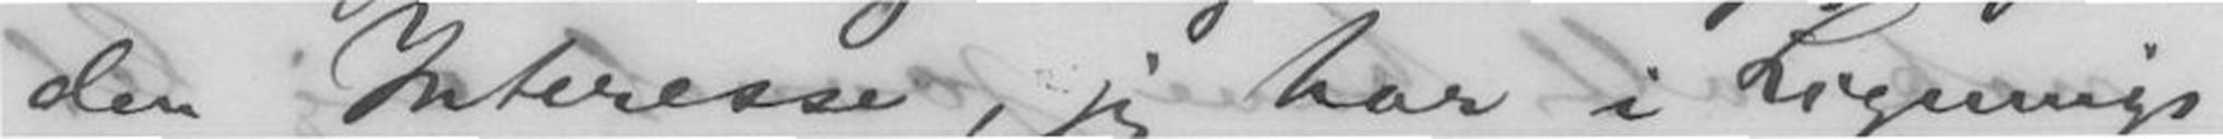den Interesse, jeg har i Lignings-
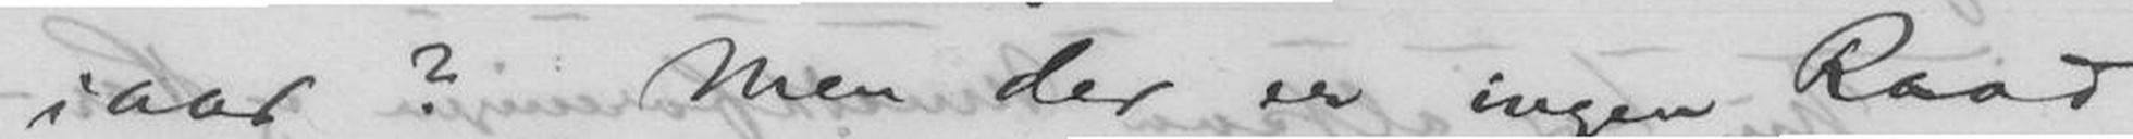iaar? Men der er ingen Raad
